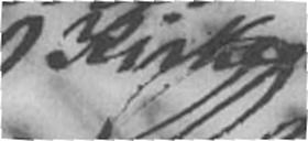Kirke
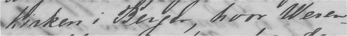kirken i Bergen, hvor Weren-
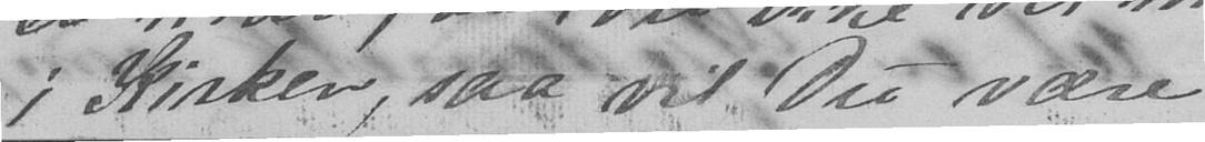i Kirken, saa vil Du være
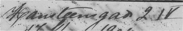Hansteensgade 2 IV
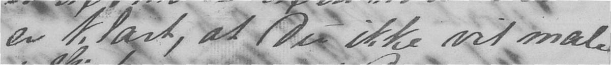er klart, at Du ikke vil male
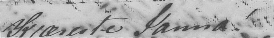Kjæreste Janna!
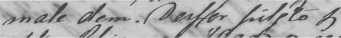male dem. Derfor fulgte jeg
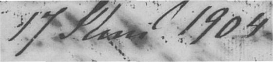17 Juni 1904.
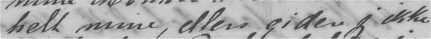helt mine, ellers gider jeg ikke
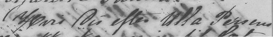Hvis Du efter Ulla Persens
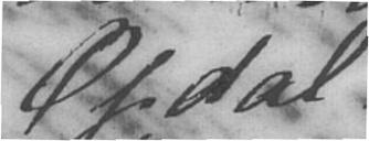Opdal
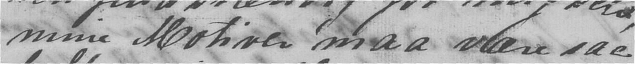mine Motiver maa være saa
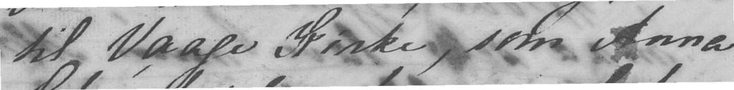til Vaage Kirke, som Anna
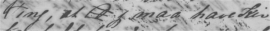Ting, at D jeg maa have Kir-
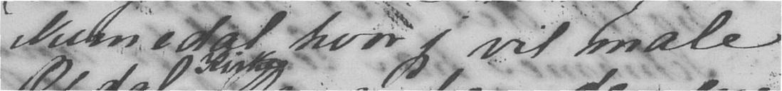Numedal hvor jeg vil male
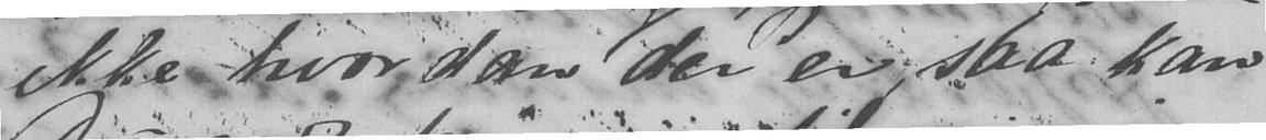ikke hvordan der er, saa kan
